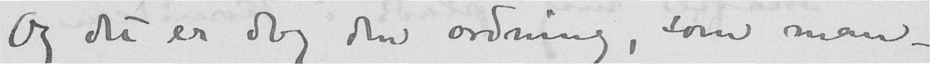Og det er dog den ordning, som man -
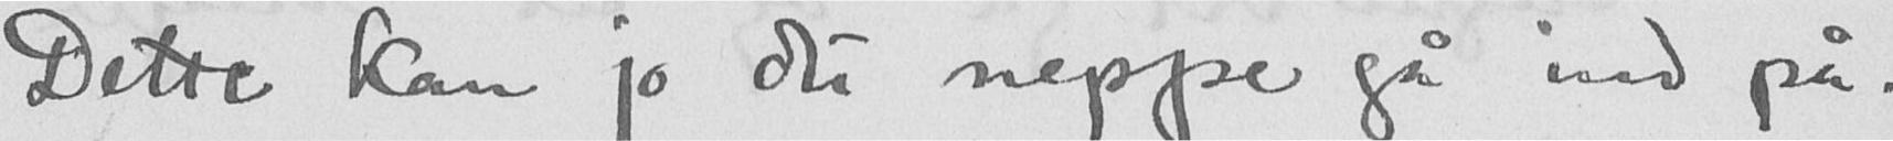Dette kan jo du neppe gå ind på.
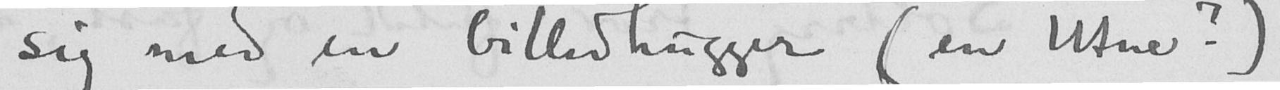sig med en billedhugger (en Utne?)
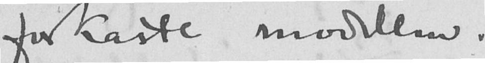forkaste modellen.
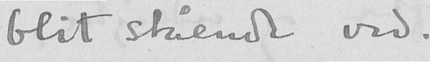blit stående ved.
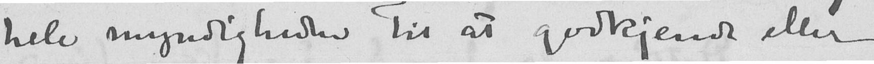hele myndigheden til at godkjende eller
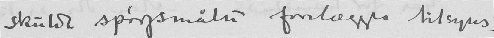skulde spørgsmålet forelægges tilsyns-
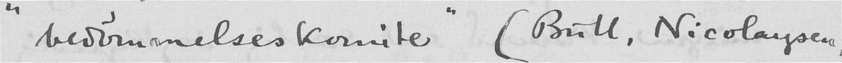"bedømmelseskomite" (Bull, Nicolaysen,
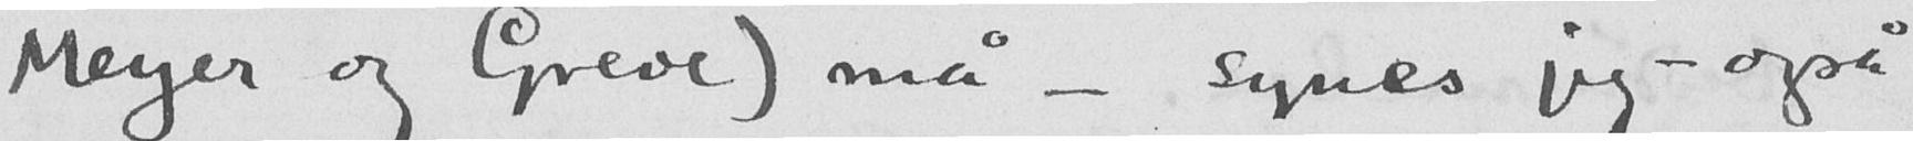Meyer og Greve) må - synes jeg - også
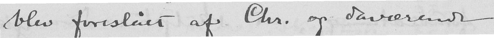blev foreslået af Chr. og daværende
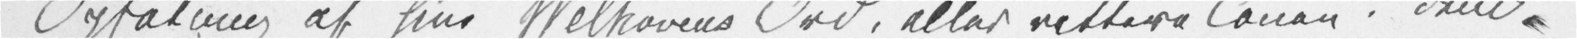Opfatning af hine Welhavens Ord, eller rettere Tonen i dem,
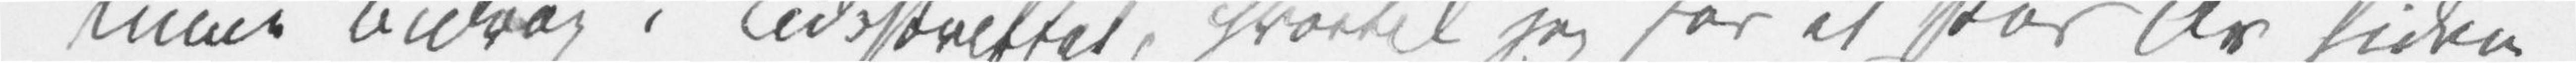mine Bidrag i Tidsskriftet, hvortil jeg for et Par År siden
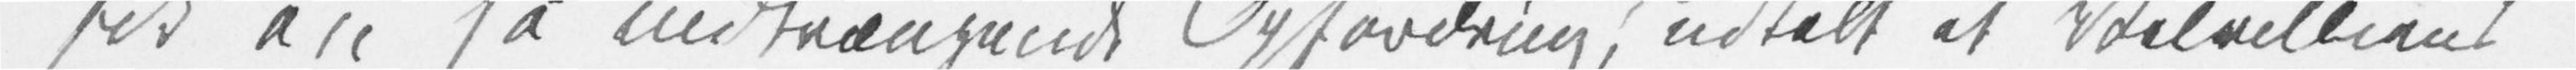fik en så indtrængende Opfordring, udtalt et Velvilliens
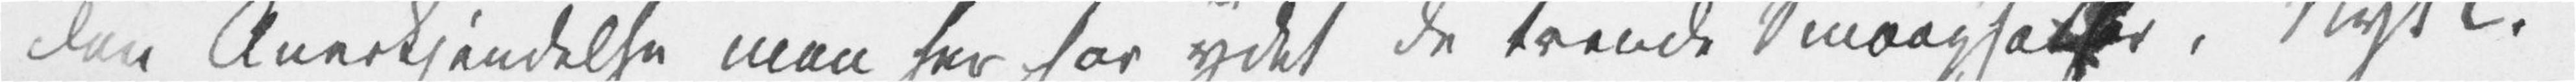den Anerkjendelse man her har ydet de tvende Småopsatser i Nyt T.
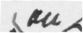en
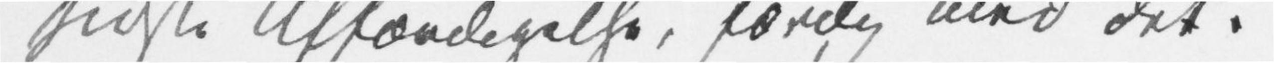sidste Affærdigelse, færdig med det.
